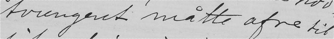tvungent måtte ofre til
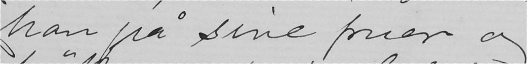han på sine fruer, og
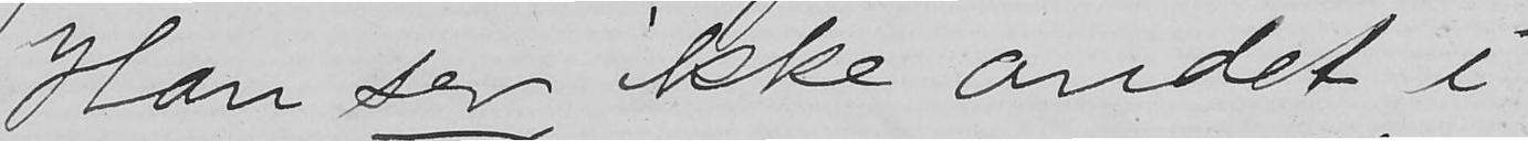Han ser ikke andet i
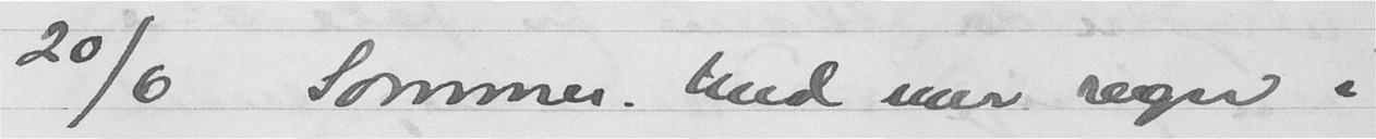20/6 Sommer med mer regn i
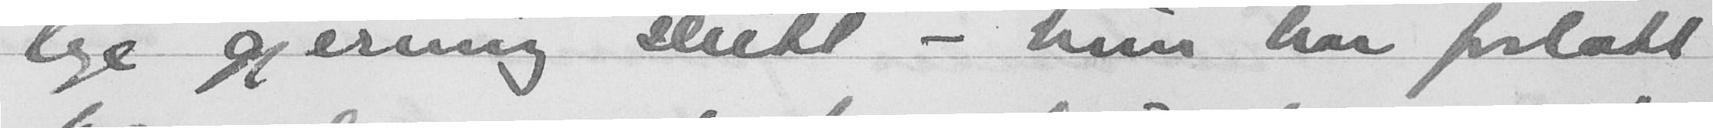lige gjerning slutt - hun har forlatt
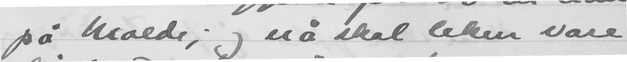på Molde; og nå skal leken vare
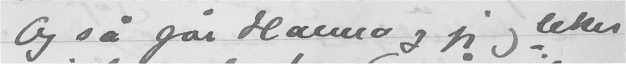Og så går Hanna og jeg og leker
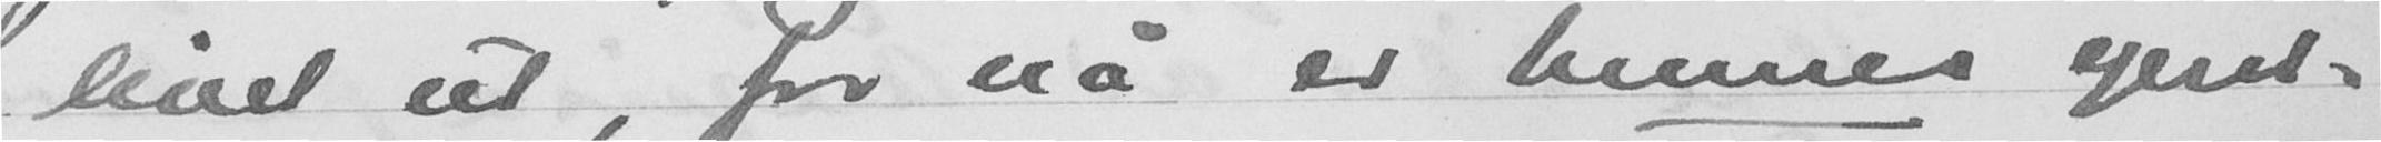livet ut, for nå, er hennes egent-
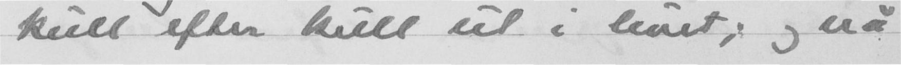kull efter kull ut i livet, og nå
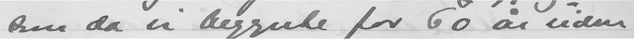som da vi begynte for 60 år siden
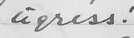ugress!
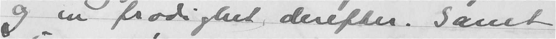og en frodighet derefter. Samt
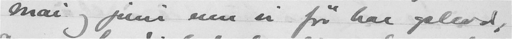mai og juni enn vi før har oplevd,
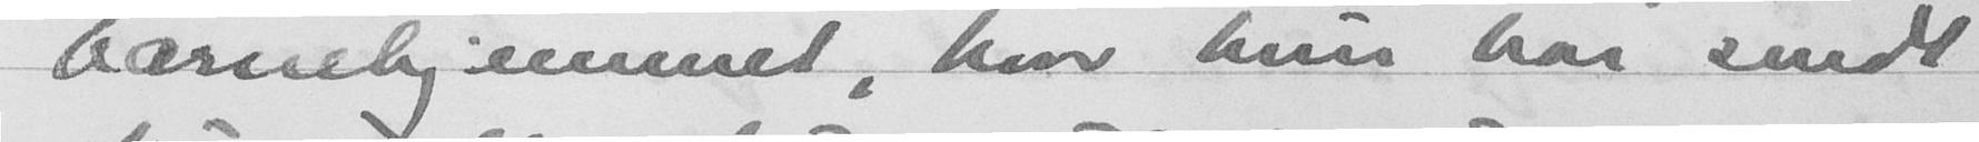barnehjemmet, hvor hun har sendt
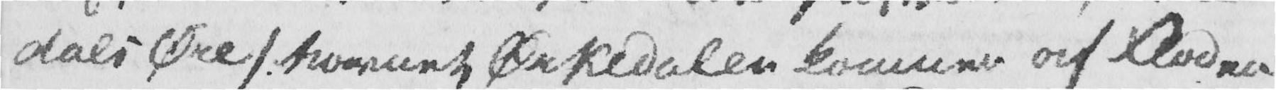dals Øre /: Navnet Ørkedalen kommer af Floden
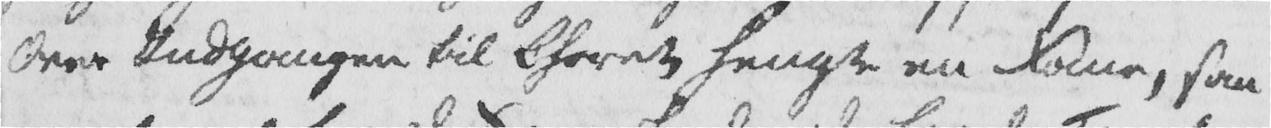Over Indgangen til Choret hengte en Fane, som
